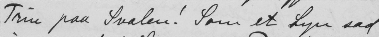Trin paa Svalen! Som et Lyn sad
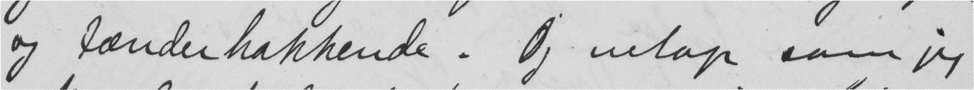og tænderhakkende. Og netop som jeg
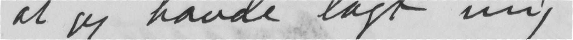at jeg havde lagt mig
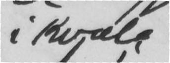i kvæld
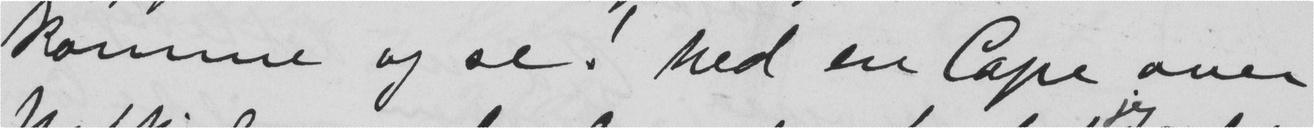komme og se. Med en Cape over
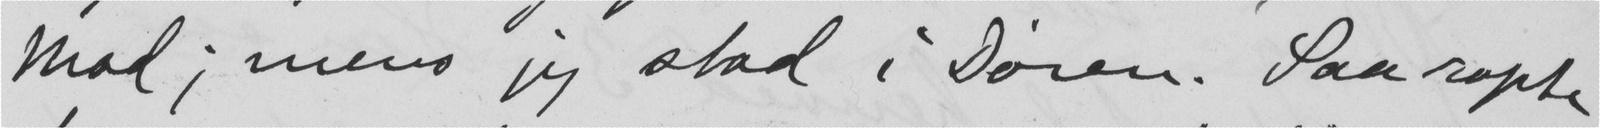Mod; mens jeg stod i Døren. Saa ropte
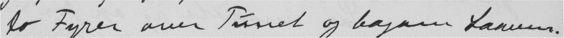to Fyrer over Tunet og bagon Laaven.
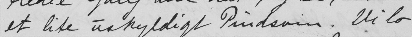et lite uskyldigt Pindsvin. Vi lo
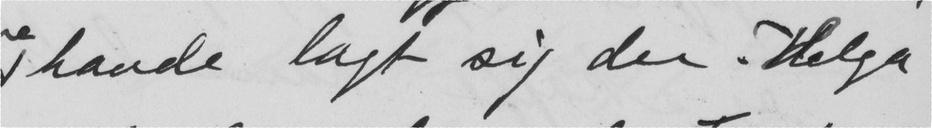havde lagt sig der. Helga
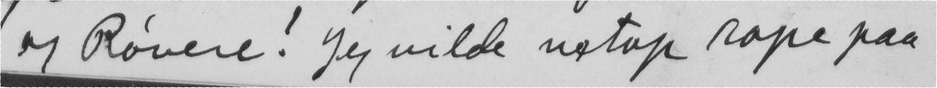og Røvere! Jeg vilde netop rope paa
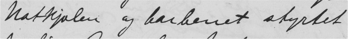Natkjolen og barbenet styrtet
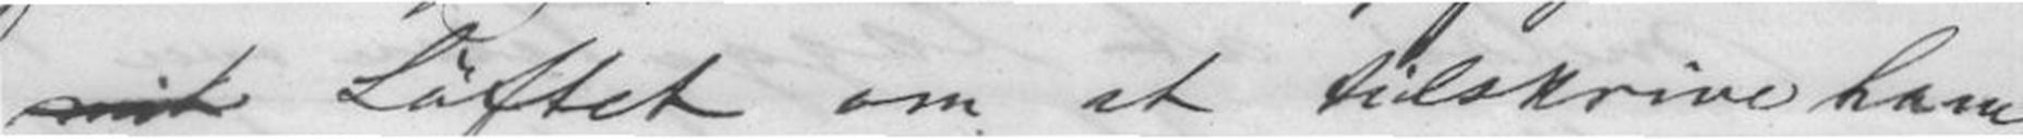mit Løftet om at tilskrive ham;
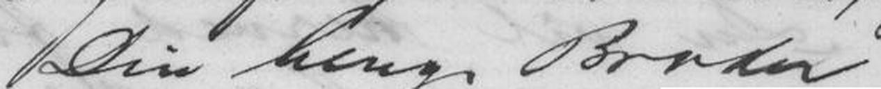Din heng. Broder
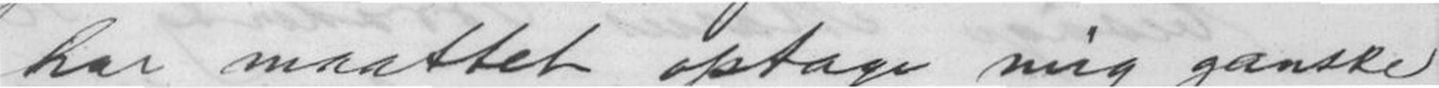har maattet optage mig ganske
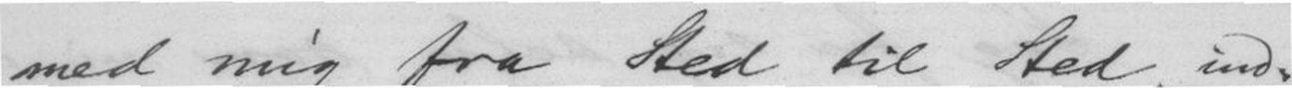med mig fra Sted til Sted, ind-
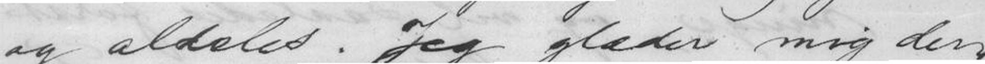og aldeles. Jeg glæder mig der-
